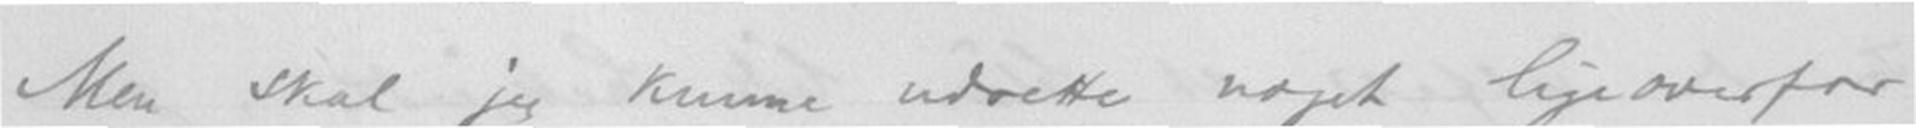Men skal jeg kunne udrette noget ligeoverfor
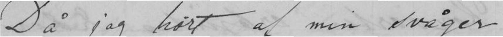Då jag hört af min svåger
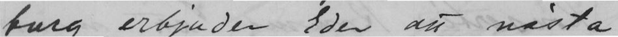burg erbjuder Eder att nästa
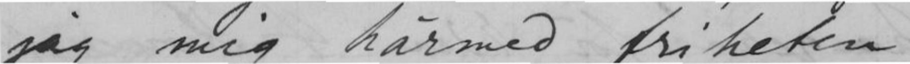jag mig härmed friheten
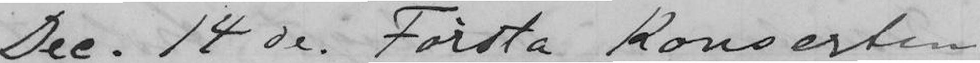Dec. 14 de. Första Konserten
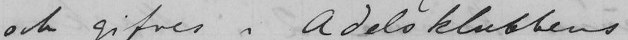och gifves i Adelsklubbens
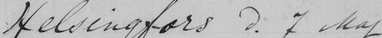Helsingfors d. 7 Maj
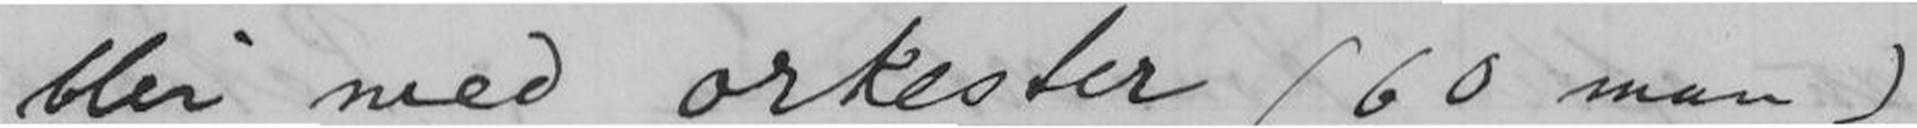blir med orkester (60 man)
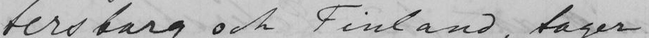tersburg och Finland, tager
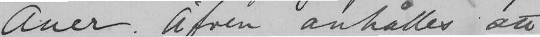Auer. Äfven anhålles att
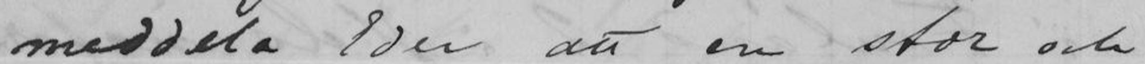meddela Eder att en stor och
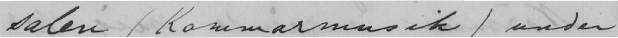salen (Kammarmusik) under
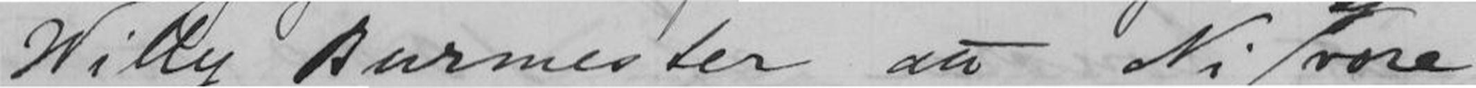Willy Burmester att Ni ej vore
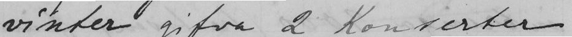vinter gifva 2 Konserter
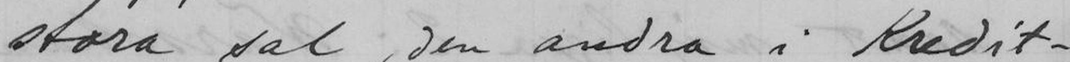stora sal, den andra i Kredit-
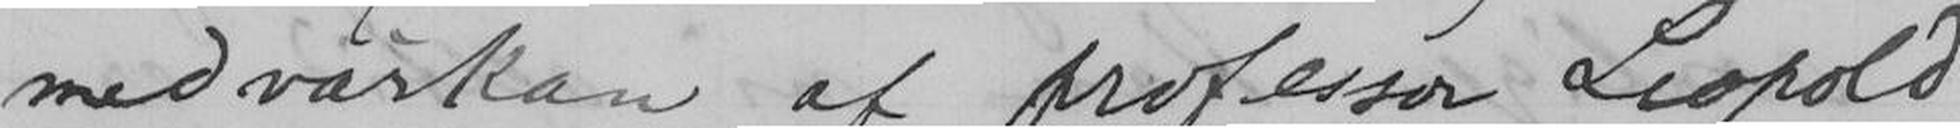medvärkan af Professor Leopold
